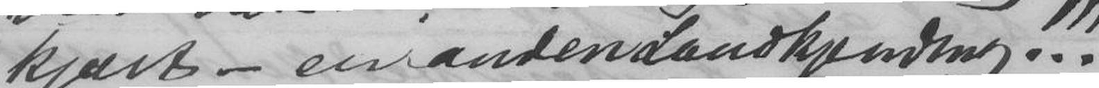kjært - en anden Landkjending!!!
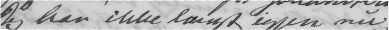Jeg har ikke langt igjen nu,
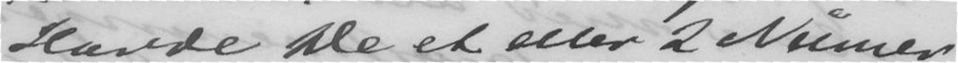Havde De et aller 2 Numer
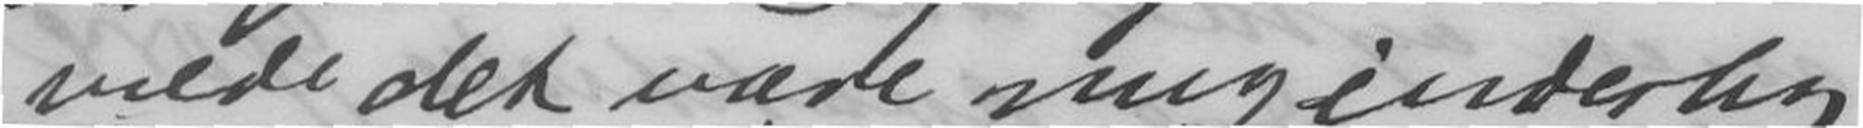vilde det være mig inderlig
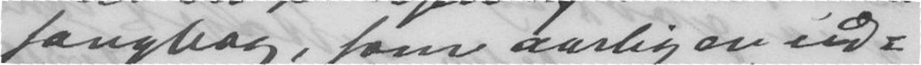sangbog, som aarlig er ud-
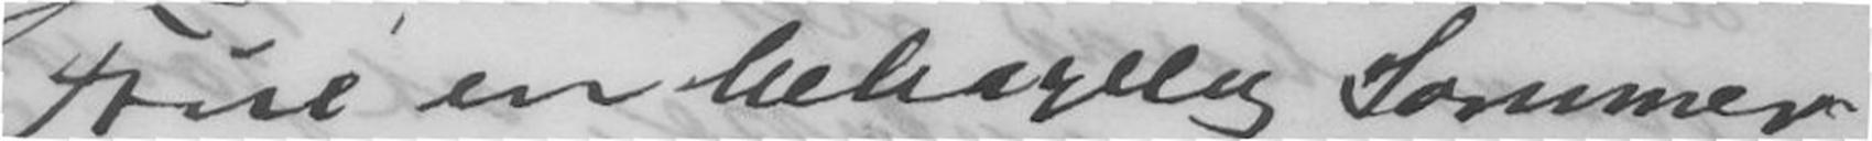Frue en behagelig Sommer
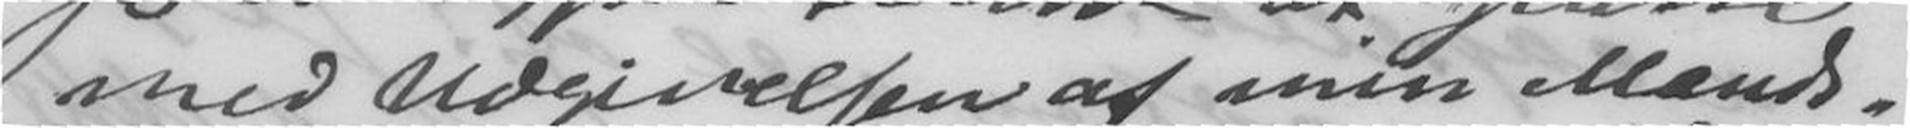med Udgivelsen af min Mands-
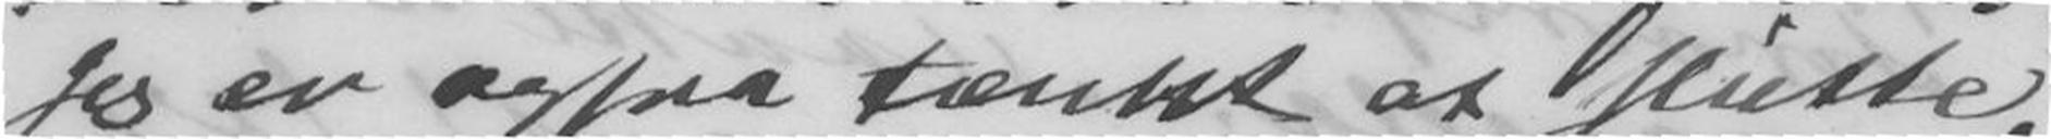Jeg er ogsaa tænkt at slutte
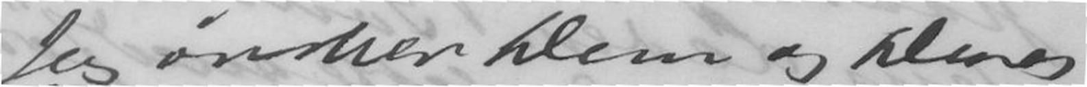Jeg ønsker Dem og Deres
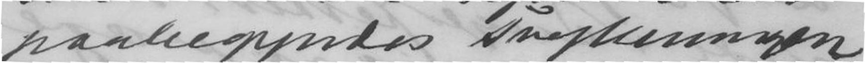paabegyndes Indskrivingen
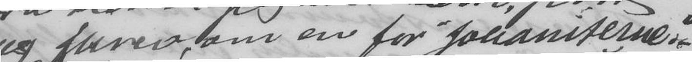jeg skrev, om en for "Johaniterne."
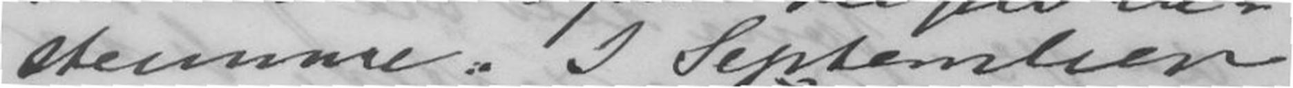stemme. I September
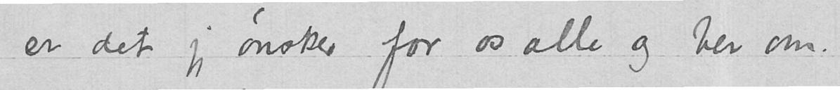er det jeg ønsker for os alle og ber om.
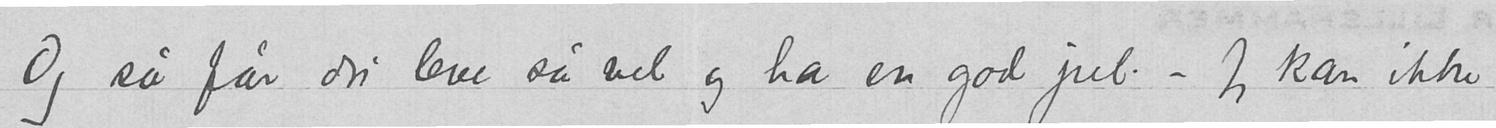Og så får du leve så vel og ha en god jul. – Jeg kan ikke
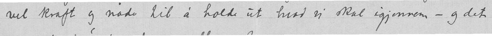vel kraft og nåde til å holde ut hvad vi skal igjennem – og det
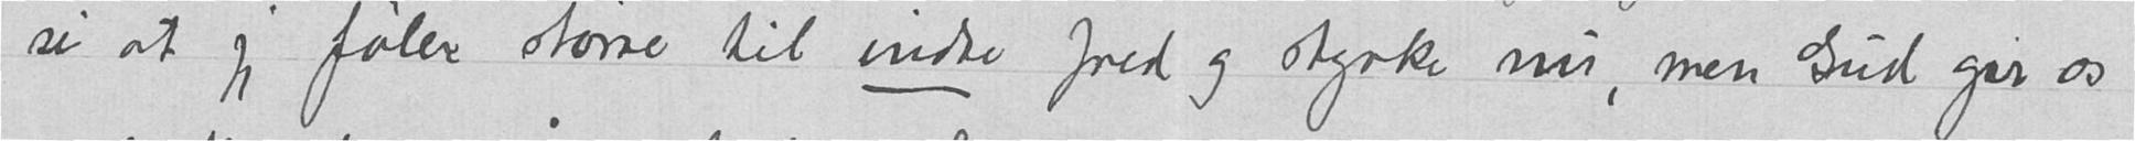si at jeg føler større til indre fred og styrke nu, men Gud gir os
